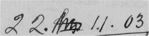22.  11. 03
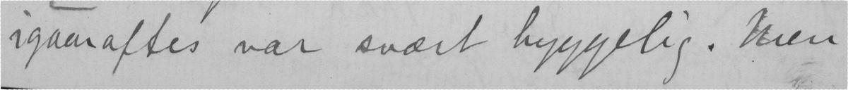igaaraftes var svært hyggelig. Men
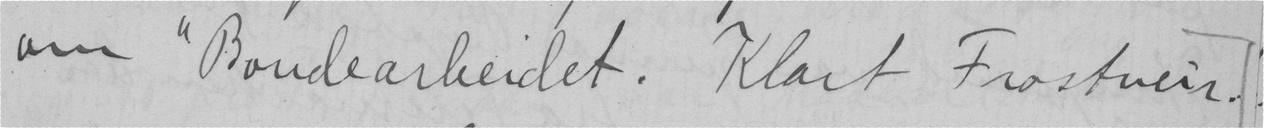om "Bondearbeidet. Klart Frostveir.
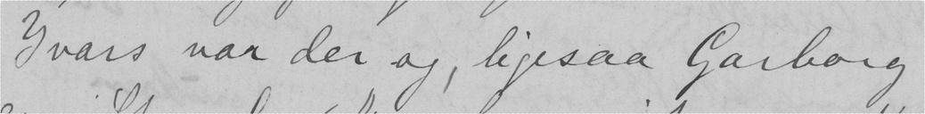Ivars var der og, ligesaa Garborg
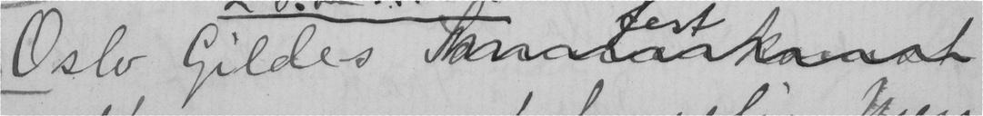Oslo Gildes
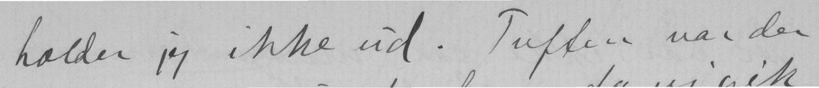holder jeg ikke ud. Tuften var der
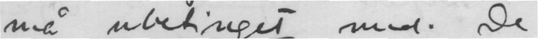må ubetinget med. De
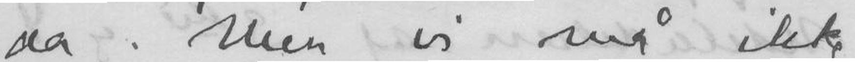da. Men vi må ikke
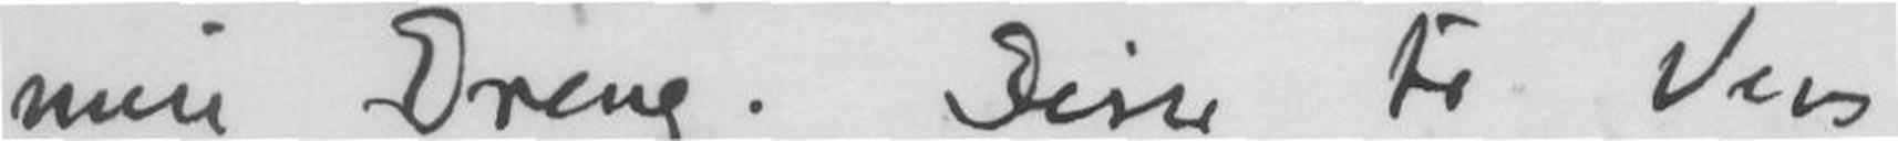min Dreng. Disse to Vers
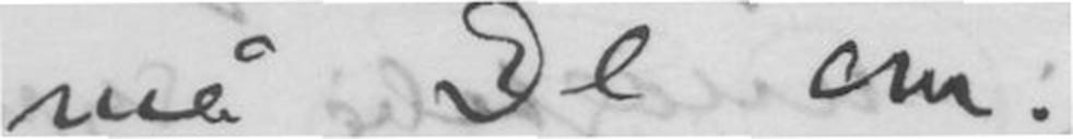må De om.
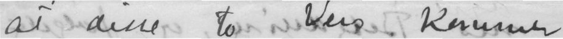at disse to Vers kommer
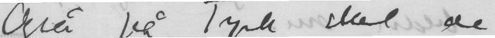Også på Tysk skal de
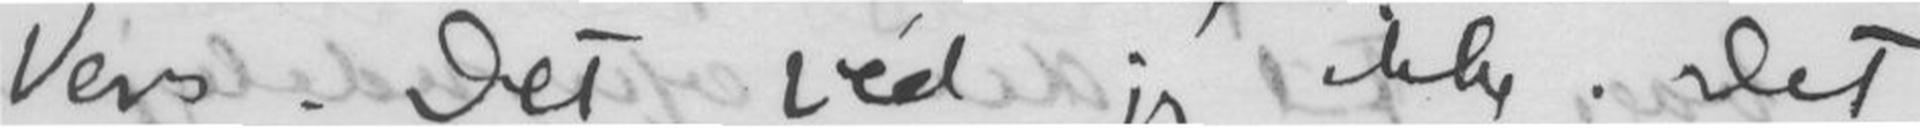Vers. Det vèd jeg ikke. Det
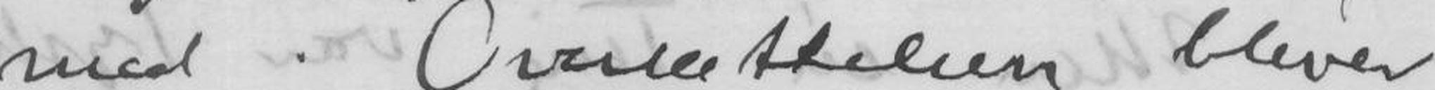med Oversættelsen bliver
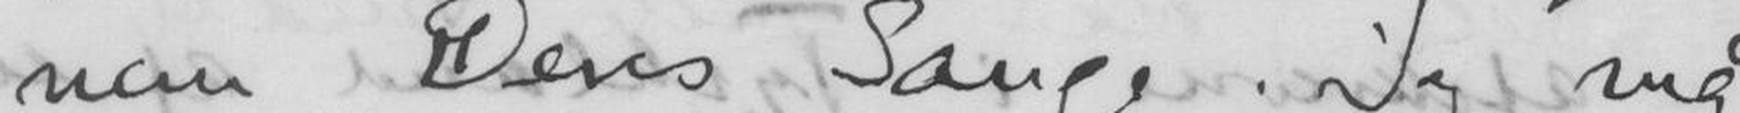nem Deres Sange. Jeg må
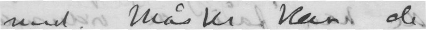med. Måske kan de
